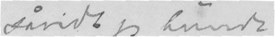såvidt jeg kunde
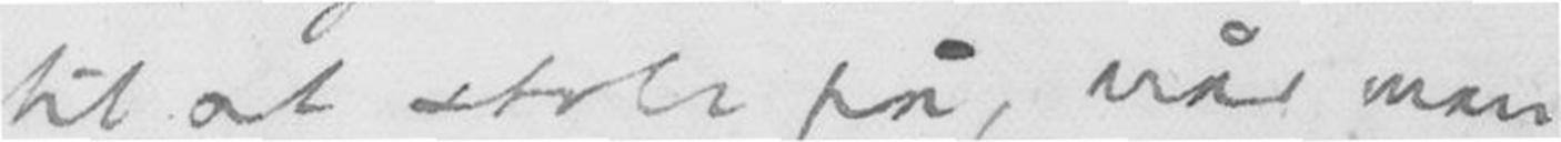til at stole på, når man
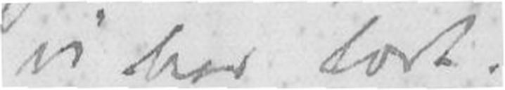vi har tort.
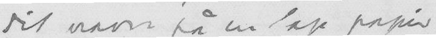dit navn på en lap papir.
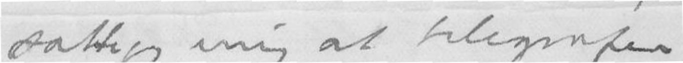satter jeg mig at telegrafere
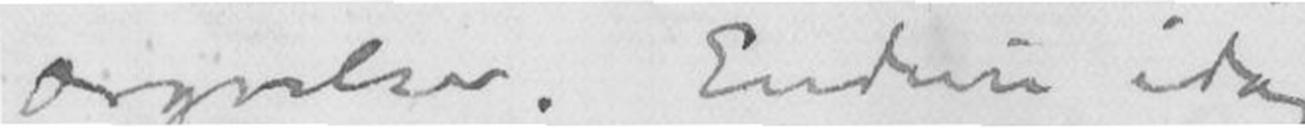ærgelser. Endnu idag
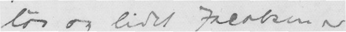løs og lidet Jacobsen er
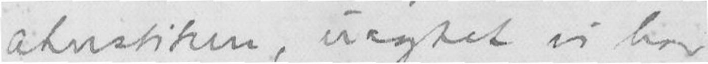akustiken, uagtet vi har
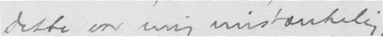Dette er mig mistænkelig,
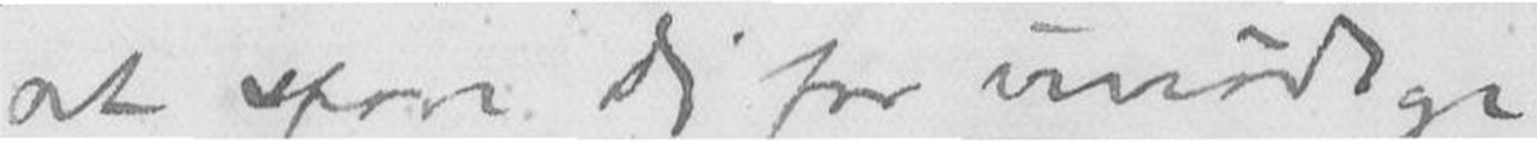at spare dig for unødige
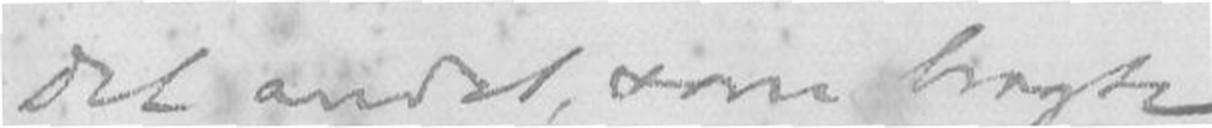Det andet, som bragte
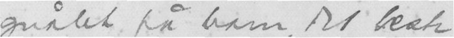gnålet på ham, det beste
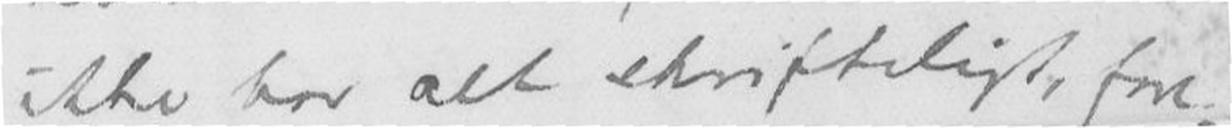ikke har alt skriftligt fore-
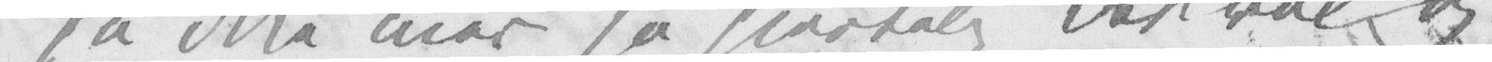så ikke mer så hjertelig Lev vel og
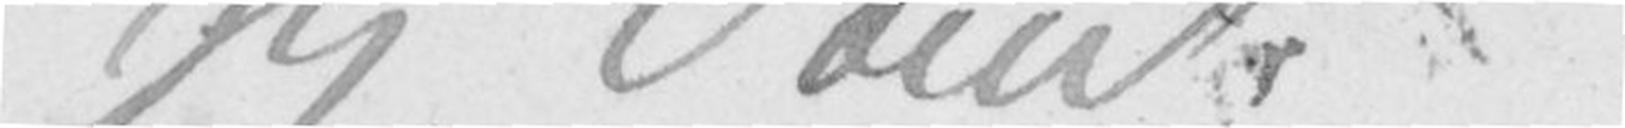jeg Dem.
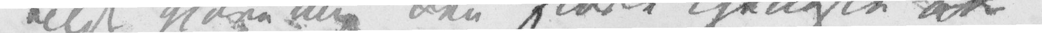vilde gjøre mig den store Tjeneste at
